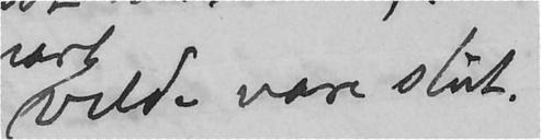vilde være slut.
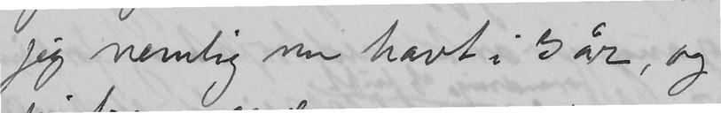jeg nemlig nu havt i 3 år, og
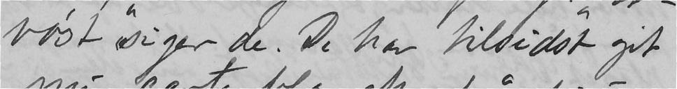vøst" siger de. De har tilsidst git
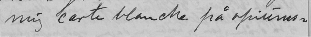mig carte blanche på opiums-
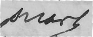snart
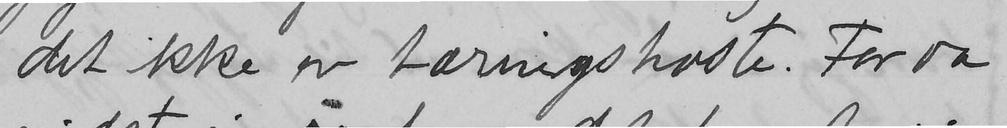det ikke er tæringshoste. For da
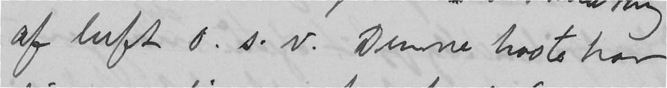af luft o.s.v. Denne hoste har
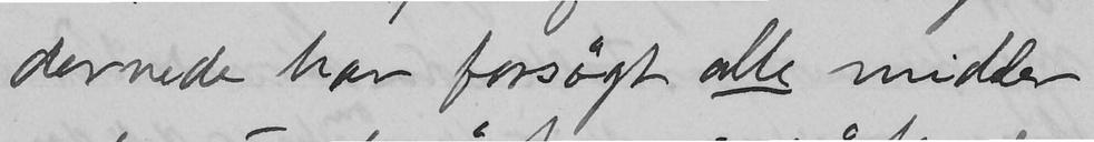dernede har forsøgt alle midler
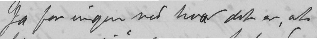Ja for ingen ved hvad det er, at
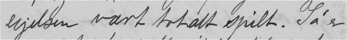lejelsen vært totalt spilt. Så er
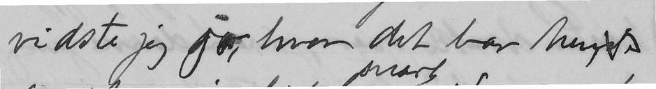vidste jeg jo, hvor det bar hen,
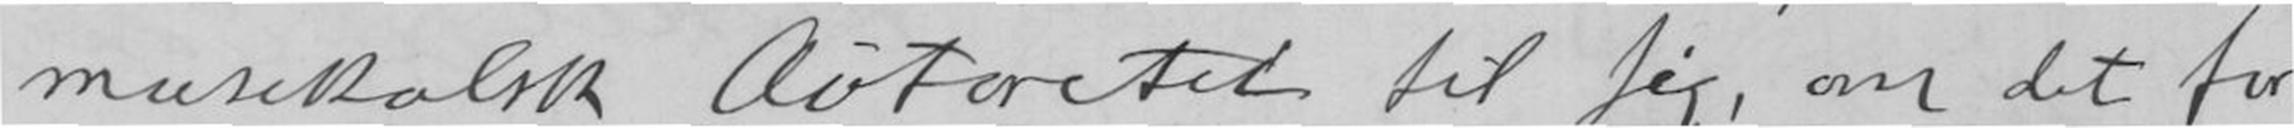musikalsk Autoritet til sig, om det for
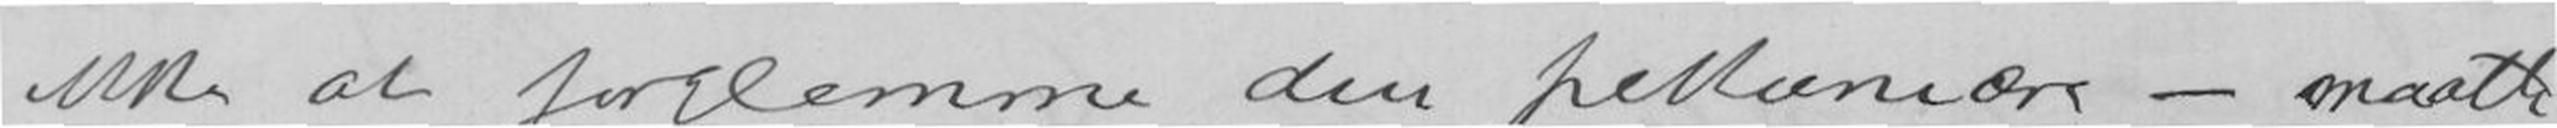ikke at forglemme den pekuniære - maatte
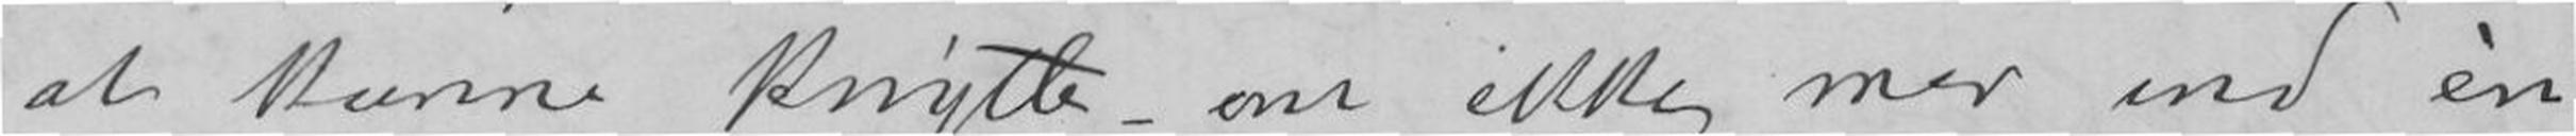at kunne knytte - om ikke mer end en
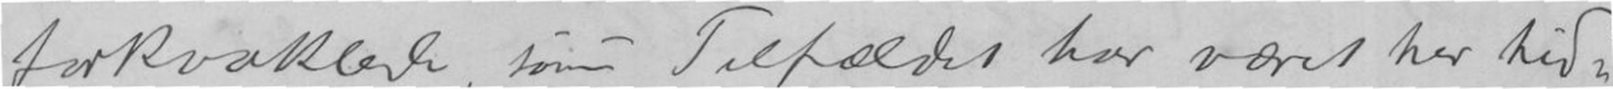forkvaklede, som Tilfældet har været her hid-
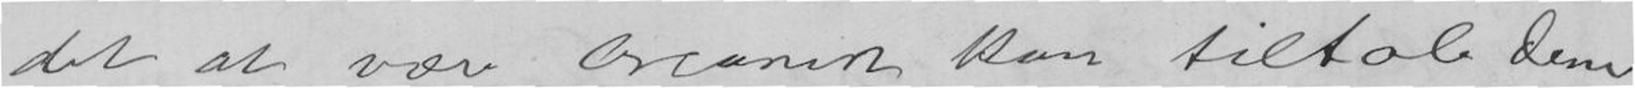det at være Organist kan tiltale Dem
